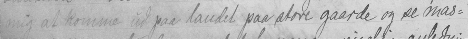mig at komme ud paa landet paa store gaarde og se mas-
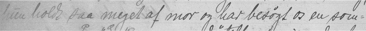hun holdt saa meget af mor og har besøgt os en som-
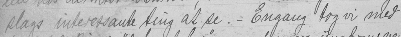slags interetsante ting at se. - Engang tog vi med
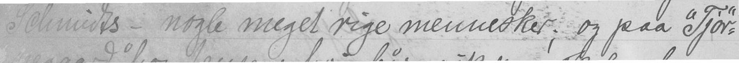Schmidts - nogle meget rige mennesker; og paa "Tjor-
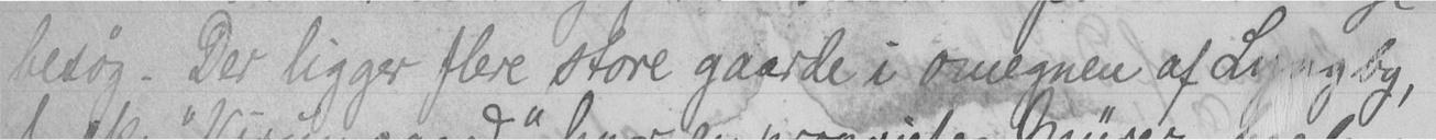besøg. Der ligger flere store gaarde i omegnen af Lyngby,
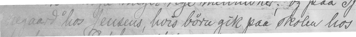negaard" hos Jensens, hvis børn gik paa skolen hos
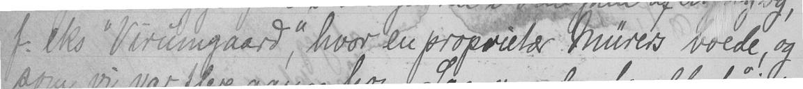f. eks "Virumgaard", hvor en proprietær Murers boede, og
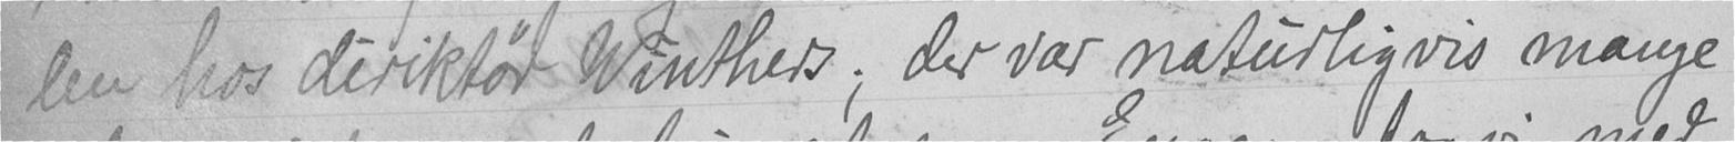len hos diriktør Winthers; der var naturligvis mange
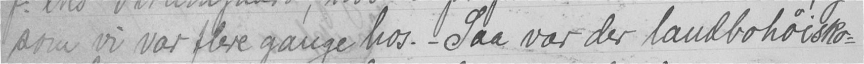som vi var flere gange hos. - Saa var der landbohøisko-
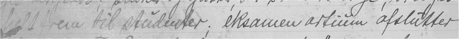helt frem til studenter; eksamen artium afslutter
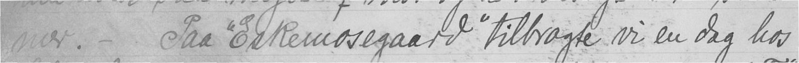mer. - Paa "Eskemosegaard" tilbragte vi en dag hos
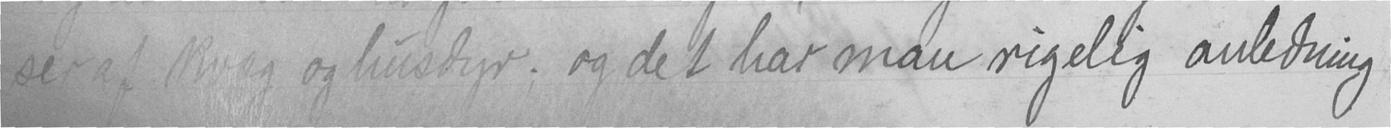ser af kveg og husdyr; og det har man rigelig anledning
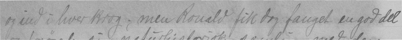og ind i hver krog; men Ronald fik dog fanget en god del
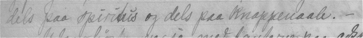dels paa spiritus og dels paa knappenaale. -
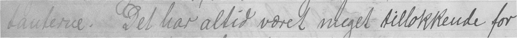tanterne. Det har altid været meget tillokkende for
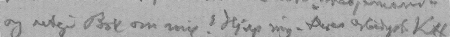og utgi Bok om mig! Hjelp mig. Deres ærbødigst KH
